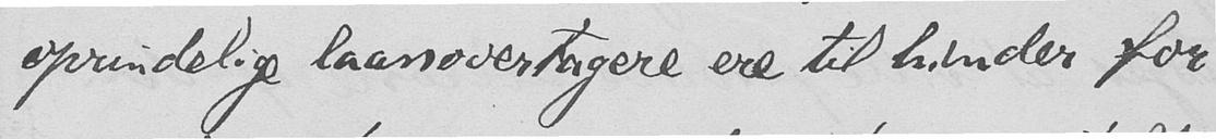oprindelige laanovertagere ere til hinder for
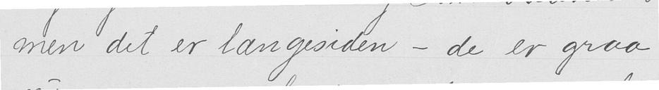men det er længesiden - de er graa
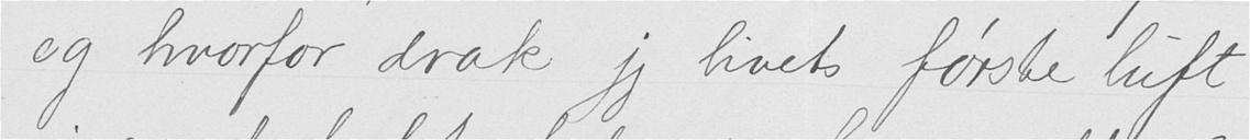og hvorfor drak jeg livets første luft
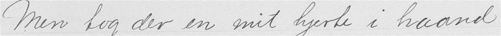Men tog der en mit hjerte i haand
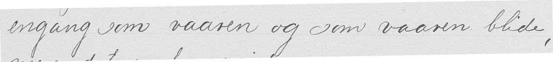engang som vaaren og som vaaren blide,
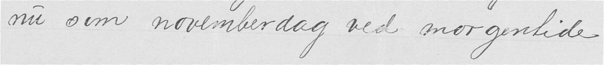nu som novemberdag ved morgentide
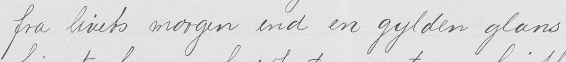fra livets morgen end en gylden glans
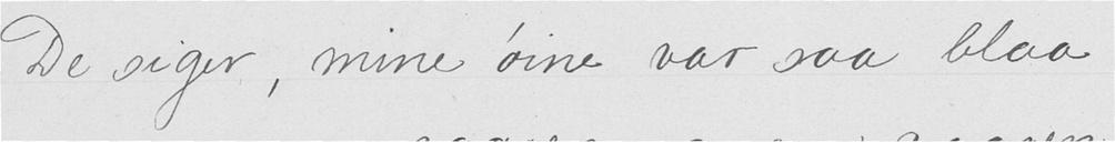De siger, mine øine var saa blaa
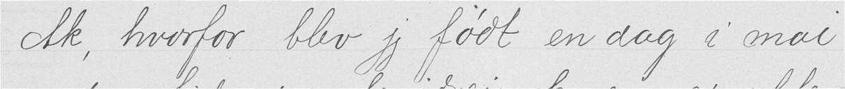Ak, hvorfor blev jeg født en dag i mai.
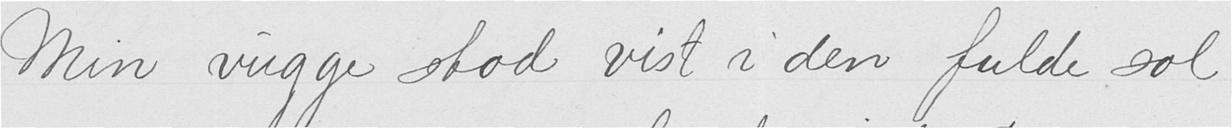Min vugge stod vist i den fulde sol -
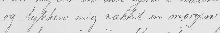og lykken mig vækket en morgen
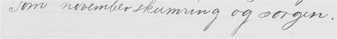som novemberskumring og sorgen.
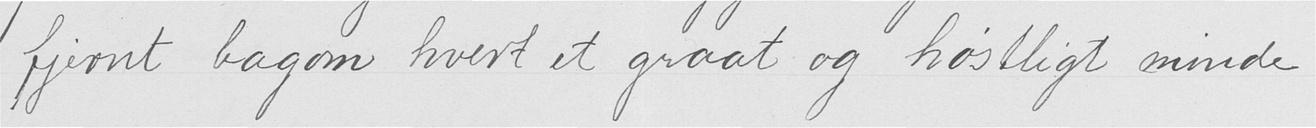fjernt bagom hvert et graat og høstligt minde.
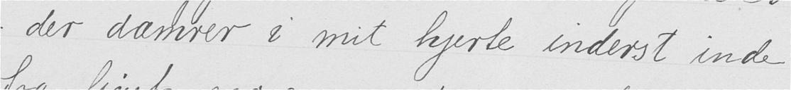- der dæmrer i mit hjerte inderst inde
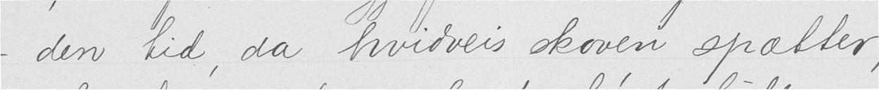- den tid, da hvidveis skoven spætter,
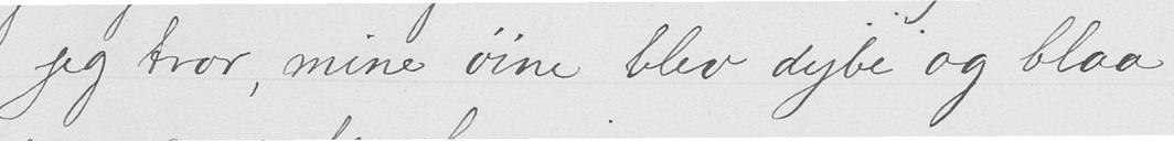- jeg tror, mine øine blev dybe og blaa
